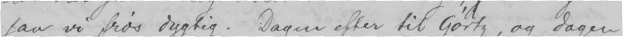saa vi frøs dygtig. Dagen efter til Gortz, og dagen
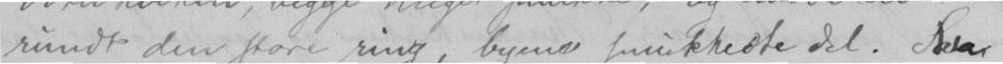rundt den store ring, byens smukkeste del.
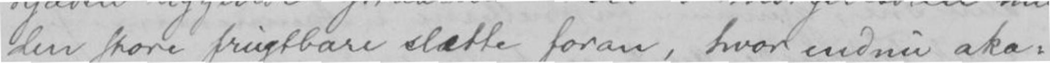den store frugtbare slætte foran, hvor endnu aka-
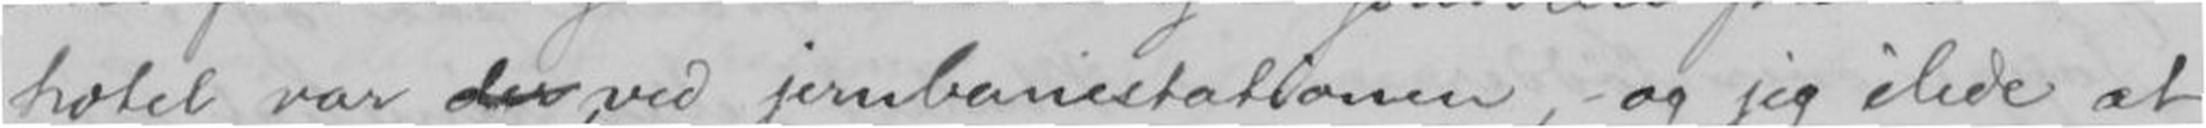hotel var ved jernbanestationen, - og jeg ilede at
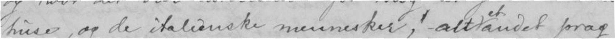huse, og de italienske mennesker, alt et andet præg
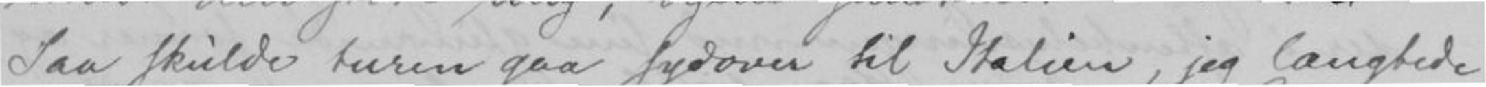Saa skulde turen gaa sydover til Italien, jeg længtede
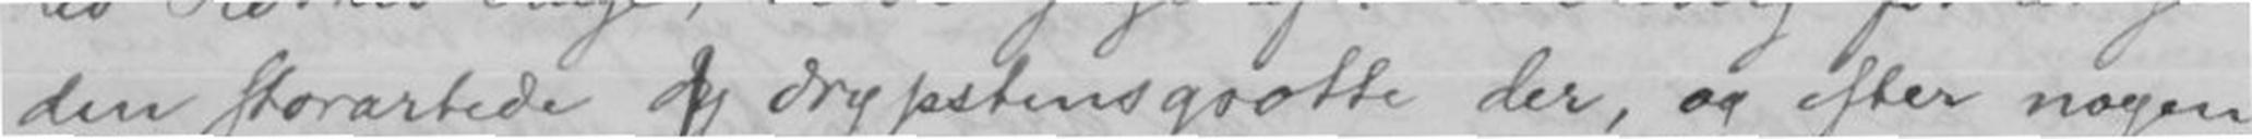den storartede drypstensgrotte der, og efter nogen
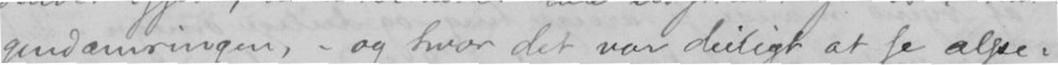gendæmringen, - og hvor det var deiligt at sa alpe-
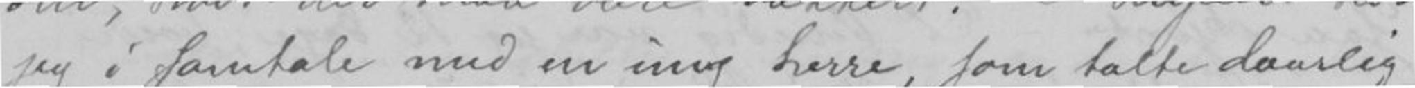jeg i samtale med en ung herre, som talte daarlig
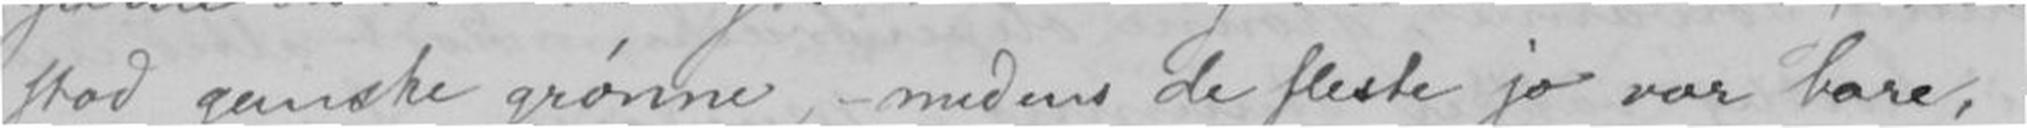stod ganske grønne, - medens de fleste jo var bare, -
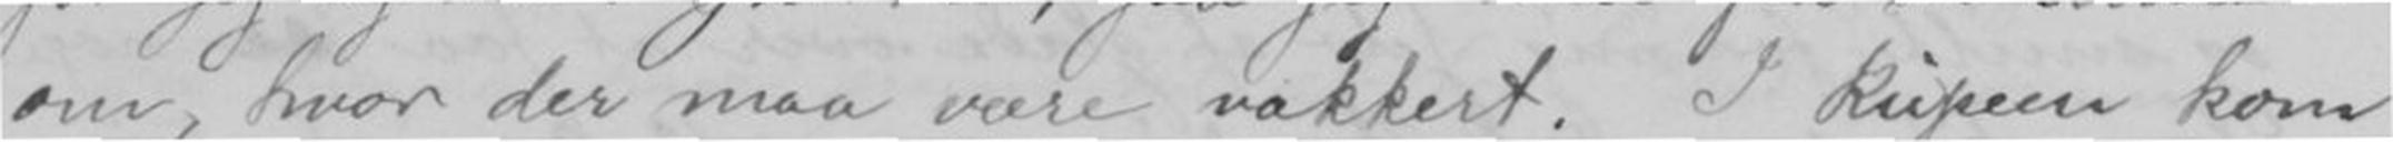om, hvor der maa være vakkert. I kupeen kom
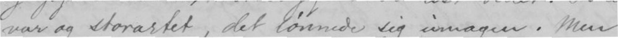var og storartet, det lønnede sig umagen. Men
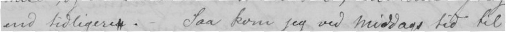end tidligere. - Saa kom jeg ved Middags tid til
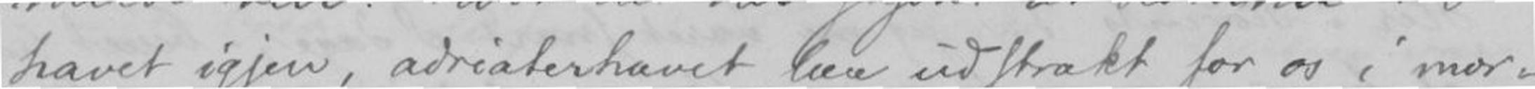havet igjen, adriaterhavet laa udstrakt for os i mor-
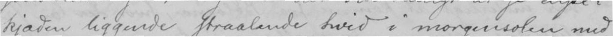kjæden liggende straalende hvid i morgensolen med
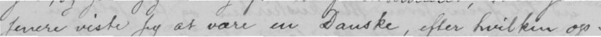senere viste sig at være en Danske, efter hvilken op-
